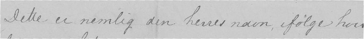Dette er nemlig den herres navn, ifølge hvis
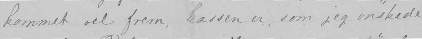kommet vel frem, kassen er, som jeg ønskede
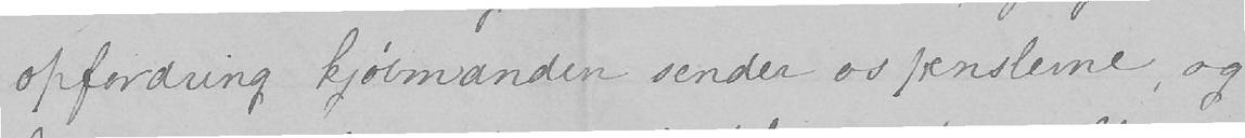opfordring kjøbmanden sender os penslerne, og
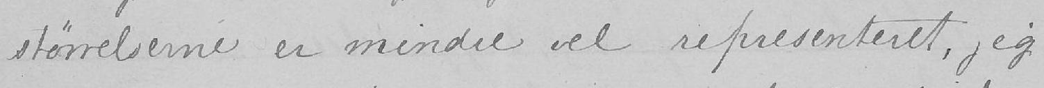størrelserne er mindre vel representeret, jeg
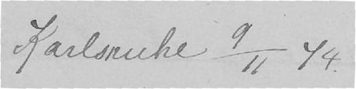Karlsruhe 9/11 74.
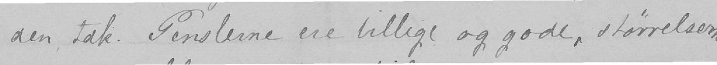den, tak. Penslerne ere billige og gode, størrelserne
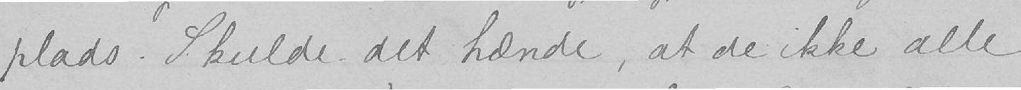plads. Skulde det hænde, at de ikke alle
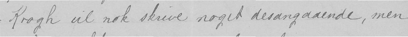Krogh vil nok skrive noget desangaaende, men
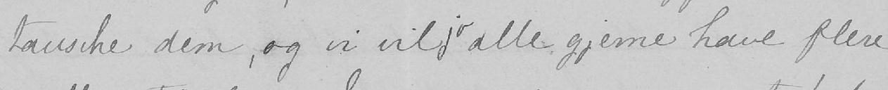tausche dem, og vi vil jo alle gjerne have flere
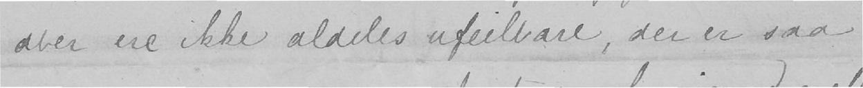aber ere ikke aldeles ufeilbare, der er saa
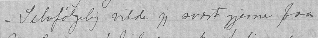— Selvfølgelig vilde jeg svært gjerne faa
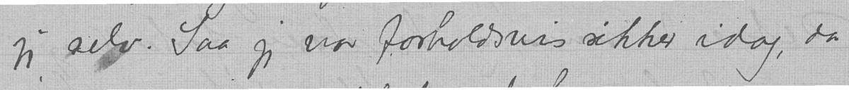jeg selv. Saa jeg var forholdsvis sikker idag, da
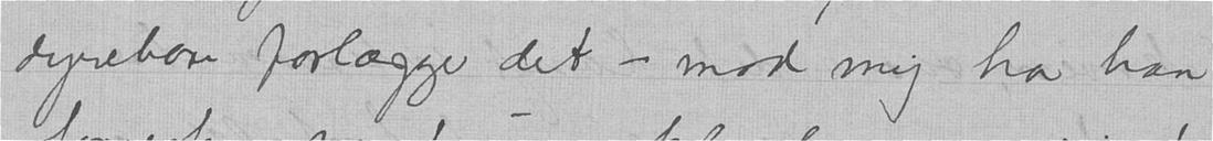dyrebare forlægger det — mod mig har han
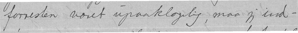forresten været upaaklagelig, maa jeg ind-
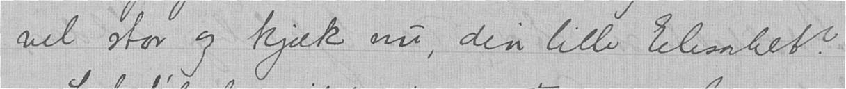vel stor og kjæk nu, din lille Elisabet?
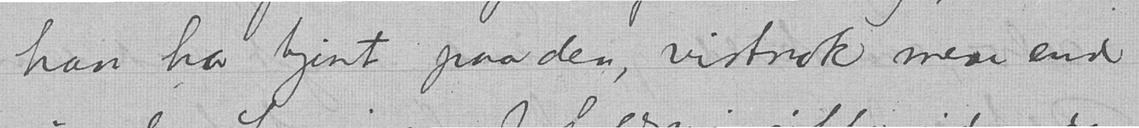han har tjent paa den, vistnok mere end
